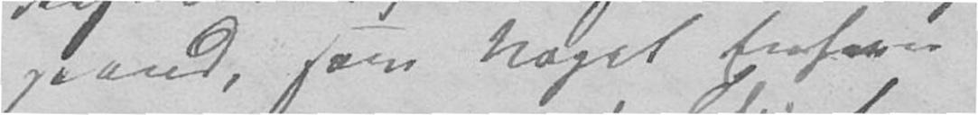stand, som Noget Enhver
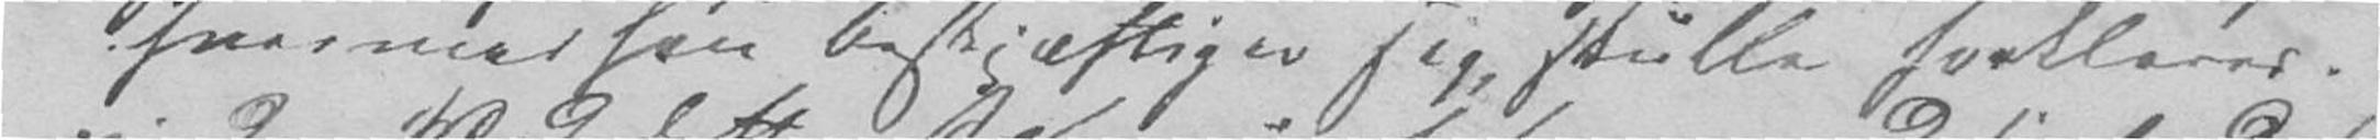hvormed han beskjæftiger sig, skulle forklares.
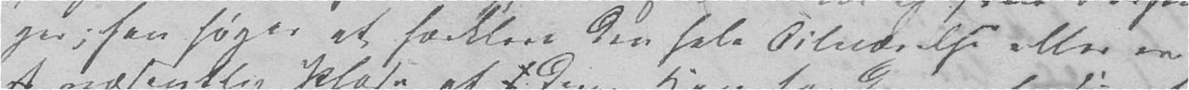ger; han søger at forklare den hele Tilværelse eller en
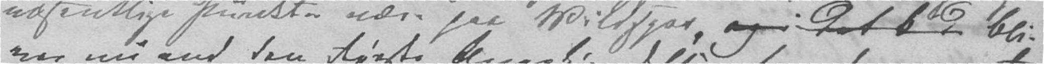væsentlige Punkter være paa Vildspor. Bli-
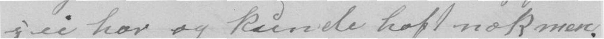jei har og kunde haft nok men
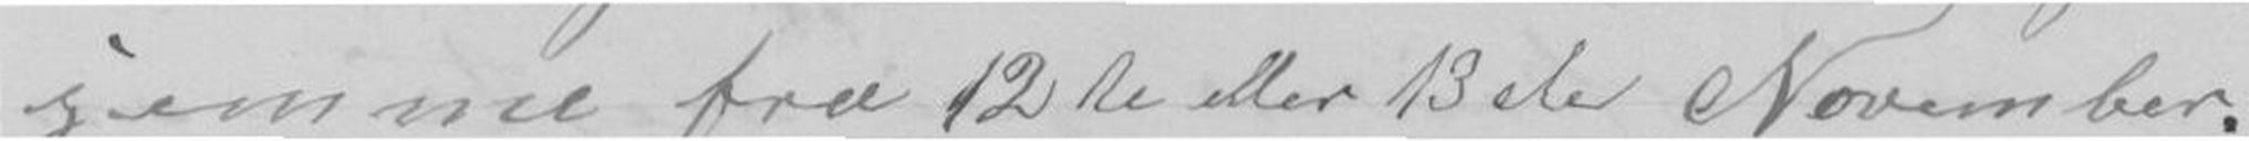jemme fra 12te eller 13de November.
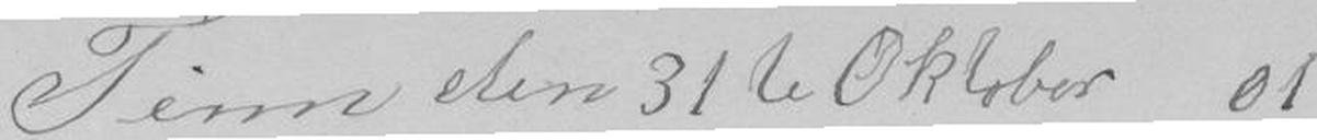Tinn den 31te Oktober 01
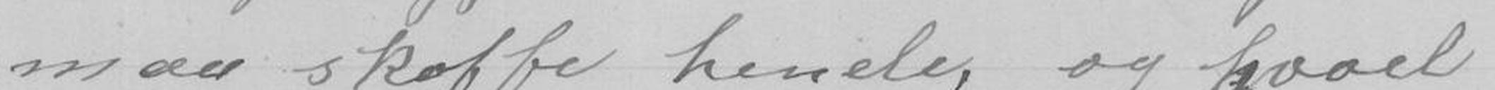maa skaffe hende, og hvad
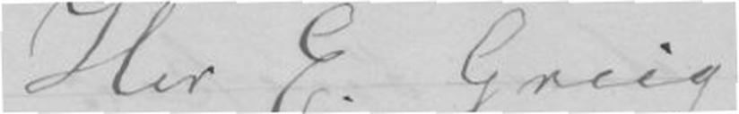Her E. Greig
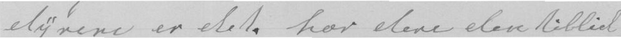dyrere er det, har dere den tillid
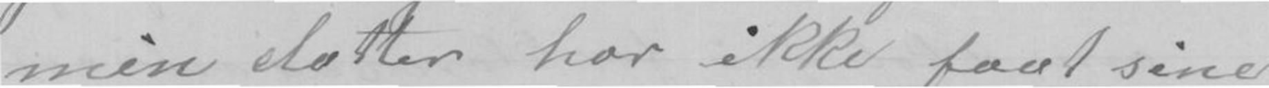min datter har ikke faat sine
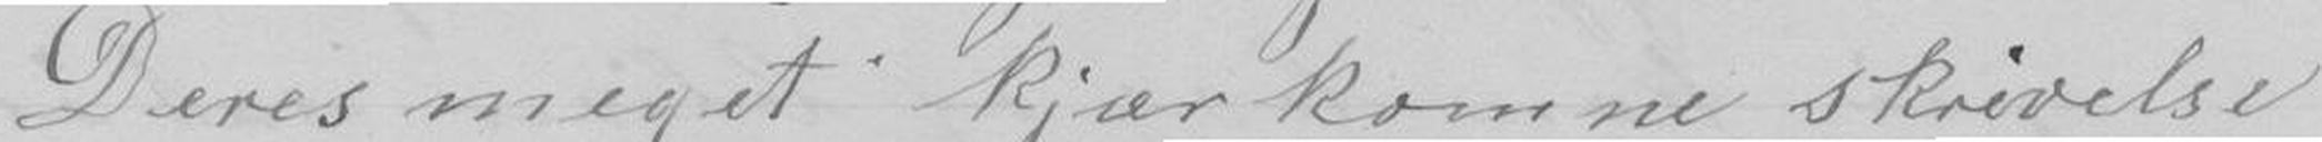Deres meget kjærkomne skrivelse
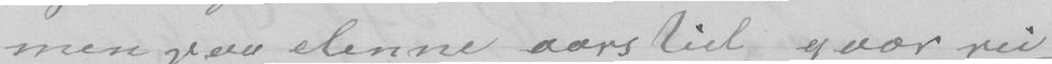men paa denne aarstid gaar rei-
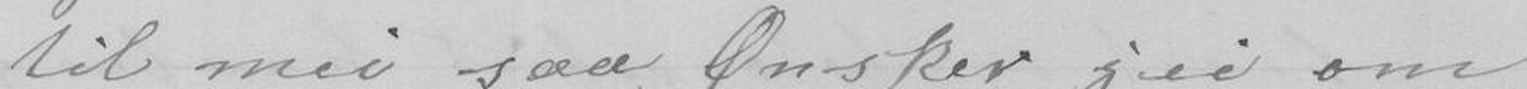til mei saa Ønsker jei om
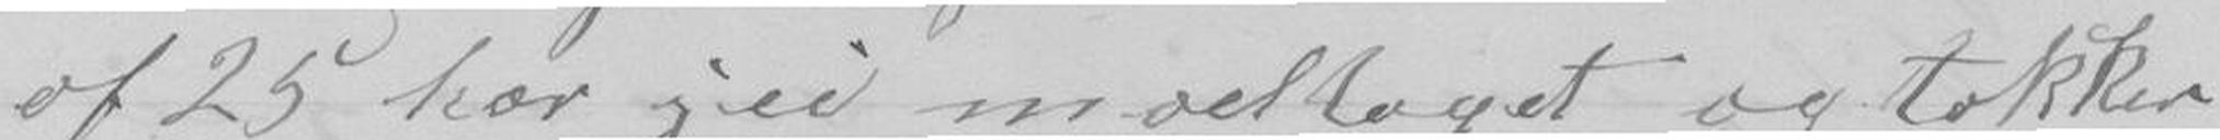af 25 har jei modtaget og takker
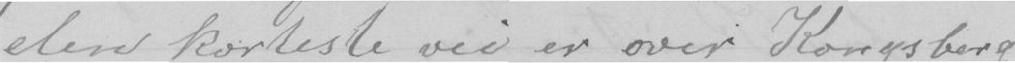den korteste vei er over Kongsberg
